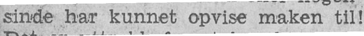sinde har kunnet opvise maken til!
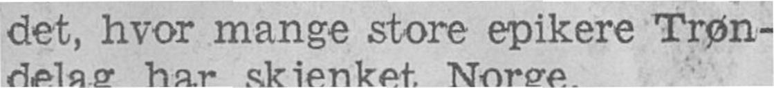det, hvor mange store epikere Trøn-
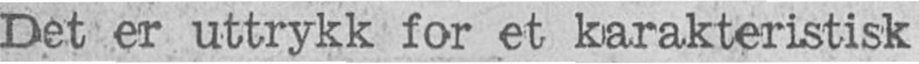Det er uttrykk for et karakteristisk
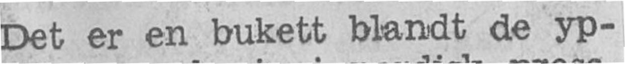Det er en bukett blandt de yp-
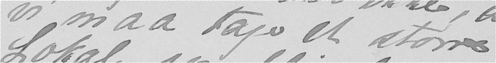vi maa tage et større
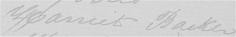Harriet Backer
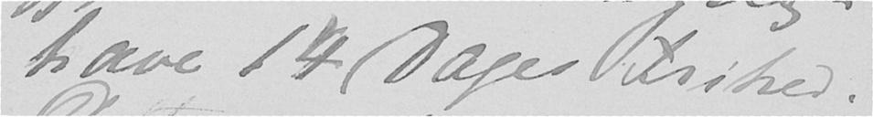have 14 Dages Frihed.
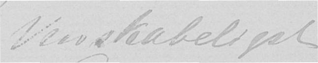Venskabeligst
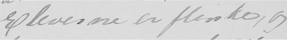Eleverne er flinke, og
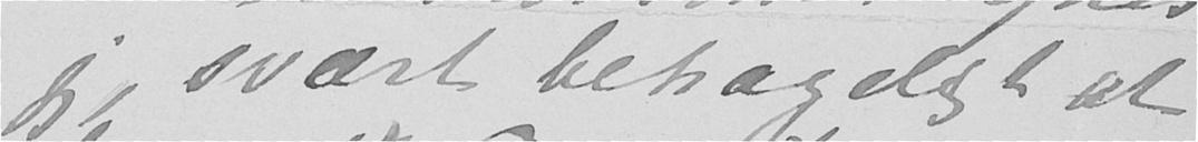jeg, svært behageligt at
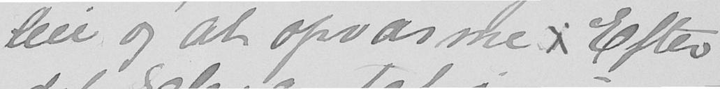leie og at opvarme. Efter
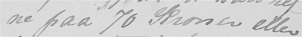ne paa 70 Kroner eller
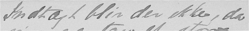Indtægt blir der ikke, da
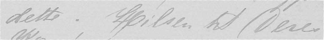dette? Hilsen til Deres
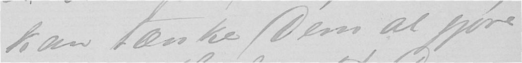kan tænke Dem at gjøre
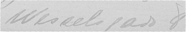Wessels gade 8
